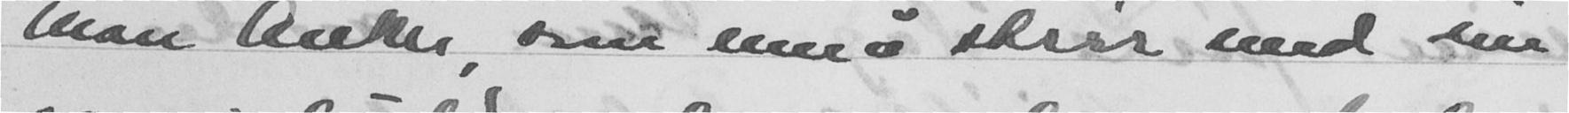Man Anker, som ennå strir med sin
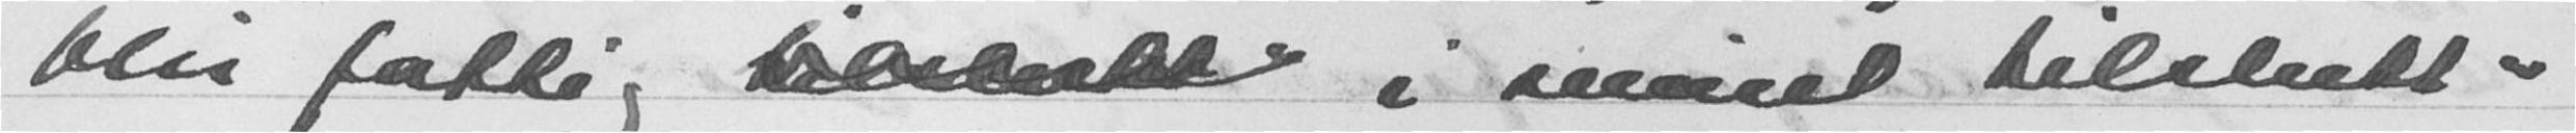blir fattig i sinnet tilslutt"
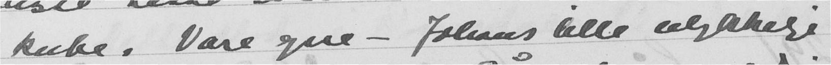kube. Vare gire - Johans lille ulykkelige
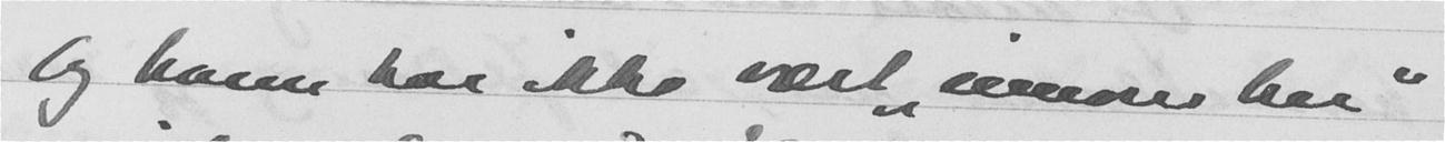Og hvem har ikke vært "innom her"
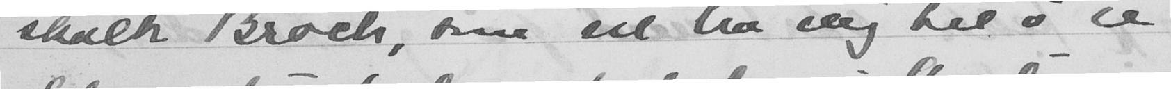skalk Broch, som vil ha mig til å se
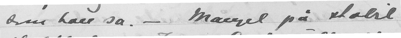som han sa. - Mangel på stabil
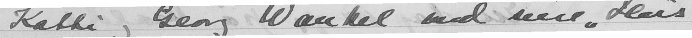Katti og Georg Wankel med sine "Heis
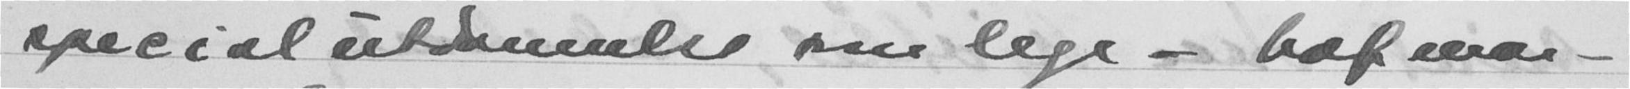specialutdannelse som lege - hofmar-
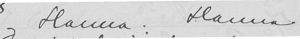og Hanna. Hanna
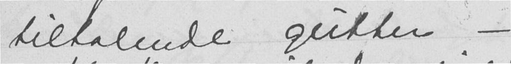tiltalende gutter -
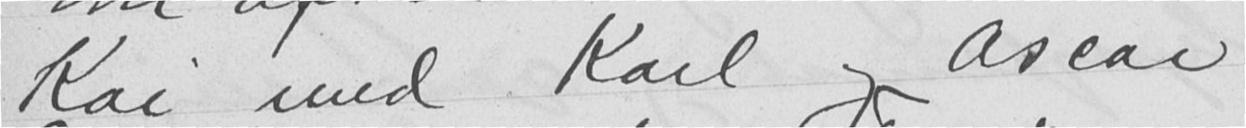Kai med Karl og Oscar
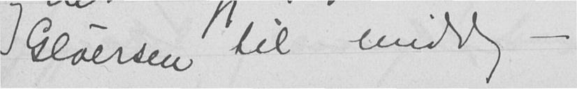Gløersen til middag -
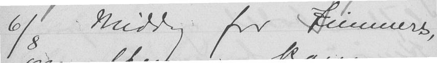6/3 Middag for Zimmers,
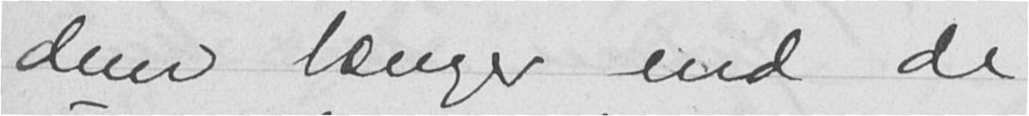dem længer end de
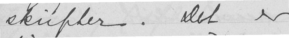skrifter. Det er
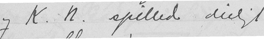og K. N. spilled deiligt
